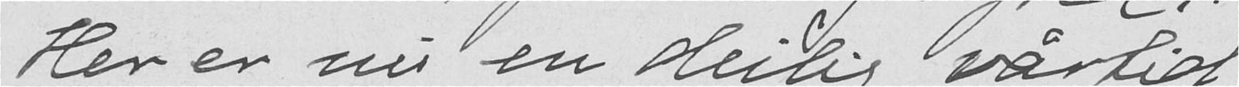Her er nu en deilig vårtid
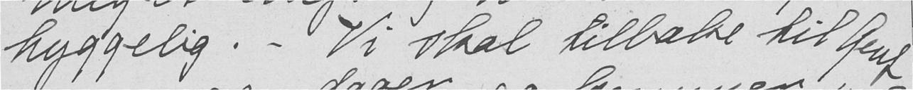hyggelig. - V skal tilbake til Gent
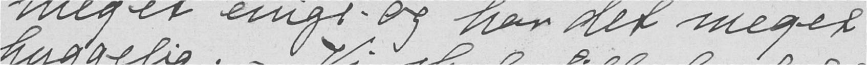meget enige og har det meget
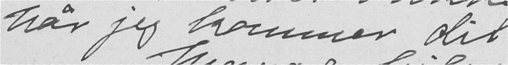når jeg kommer dit!
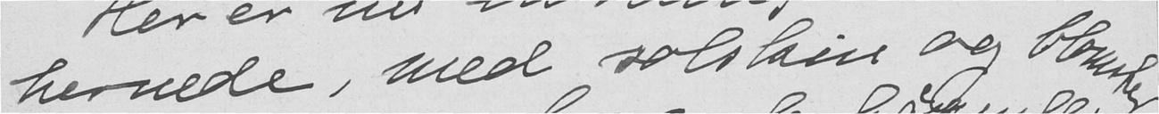hernede, med solskin og blomster
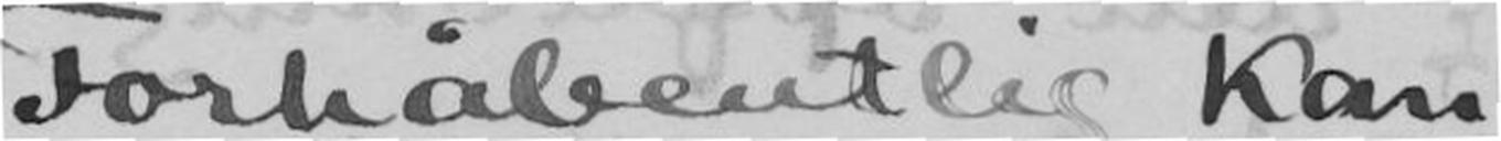Forhåbentlig kan
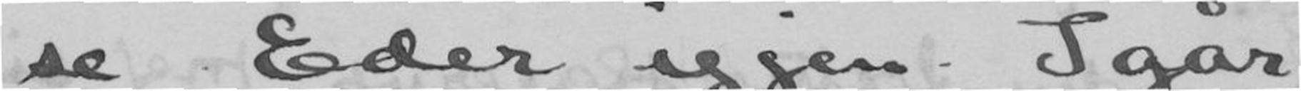se Eder igjen. Igår
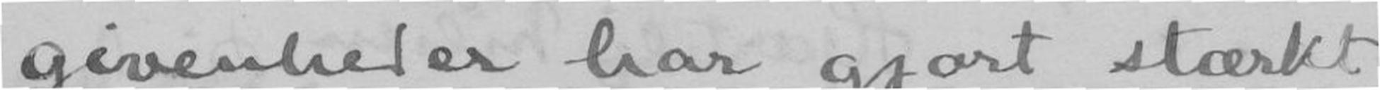givenheder har gjort stærkt
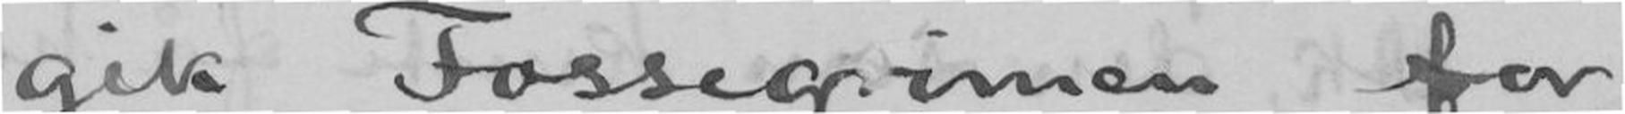gik Fossegrimen for
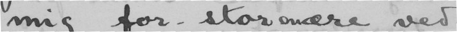mig for stor en ære ved
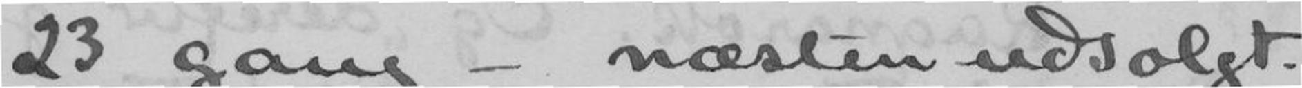23 gang - næsten udsolgt.
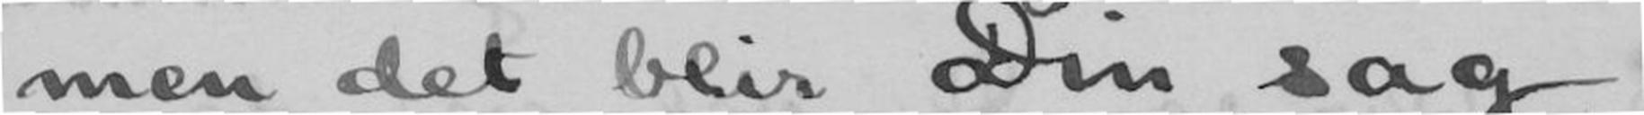men det blir Din sag.
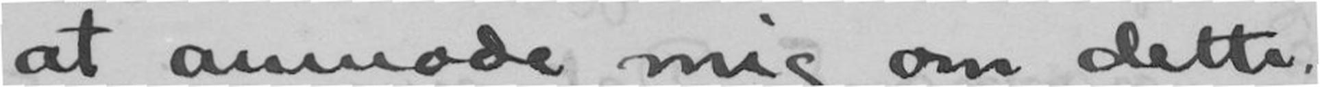at anmode mig om dette,
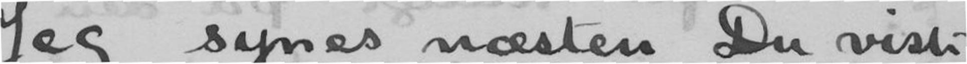Jeg synes næsten Du viste
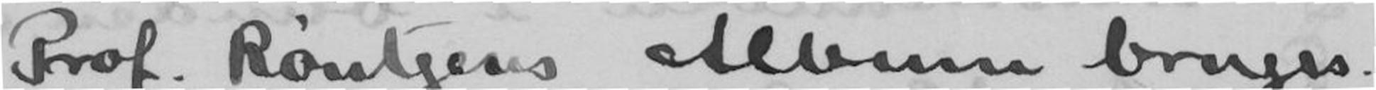Prof. Röntgens Album bruges.
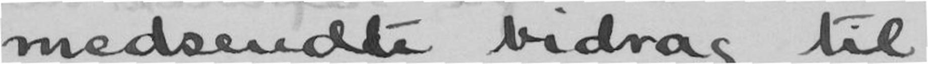medsendte bidrag til
